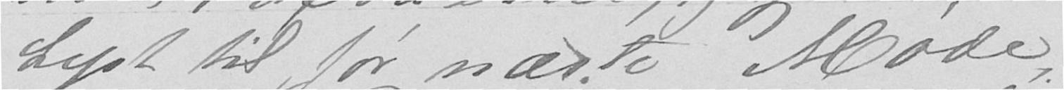Lyst til før næste Møde,
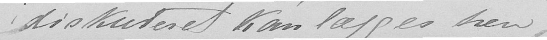diskuteret kan lægges hen
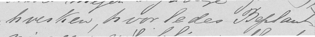hverken, hvorledes Beplant-
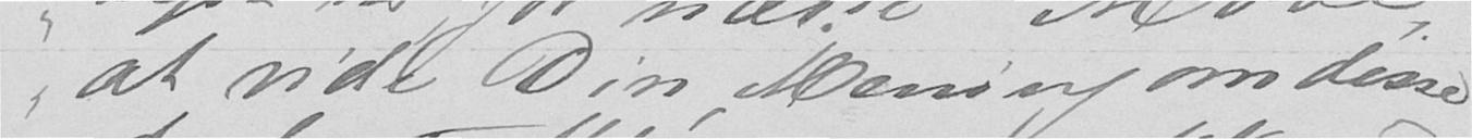at vide Din Mening om disse
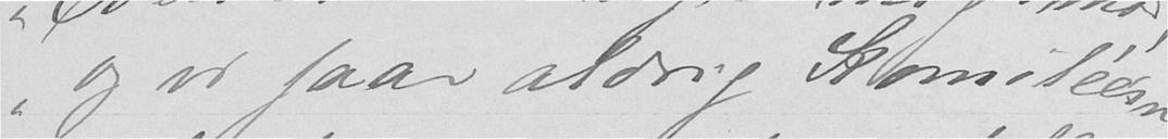og vi faar aldrig Komitéen
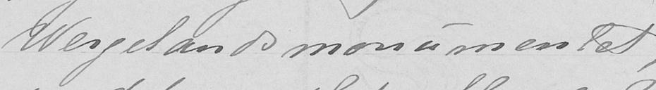Wergelandsmonumentet,
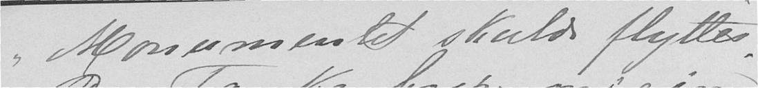Monumentet skulde flyttes.
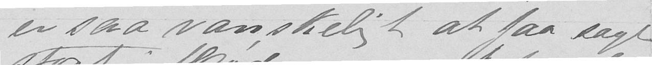er saa vanskeligt at faa sagt
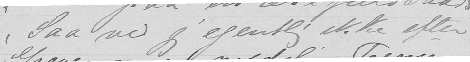Saa ved jeg egentlig ikke efter
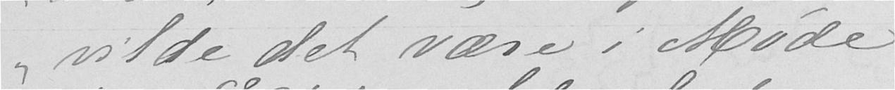vilde det være i Møde
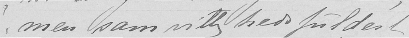men samvittighedsfuldest
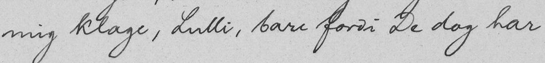mig klage, Lulli, bare fordi De dog har
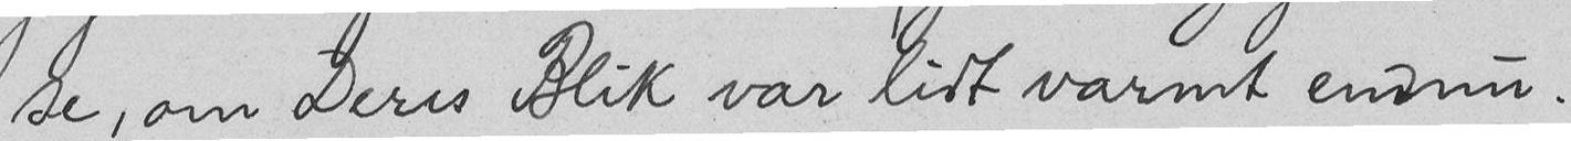se, om Deres Blik var lidt varmt endnu.
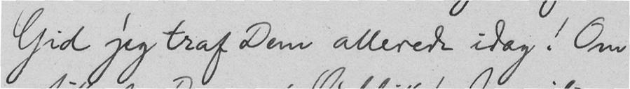Gid jeg traf Dem allerede idag! Om
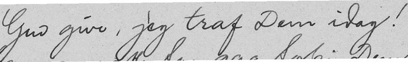Gud give, jeg traf Dem idag!
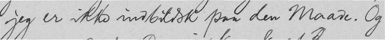jeg er ikke indbildsk paa den Maade. Og
